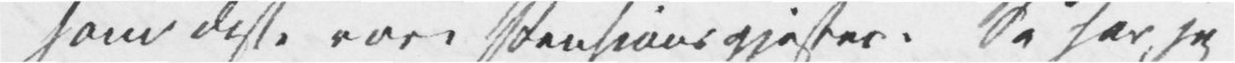som disse vore Pensionsgjester. Så har jeg
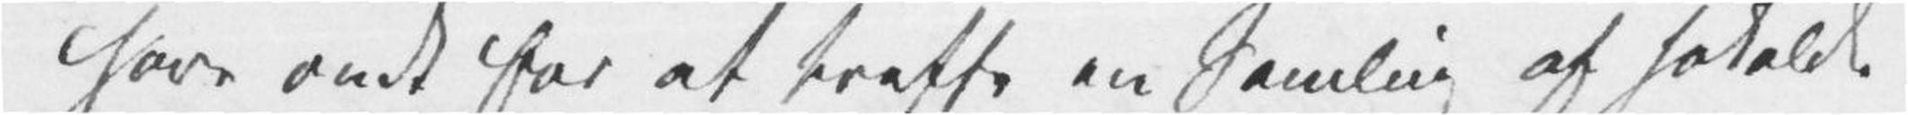have ondt for at treffe en Samling af såkaldte
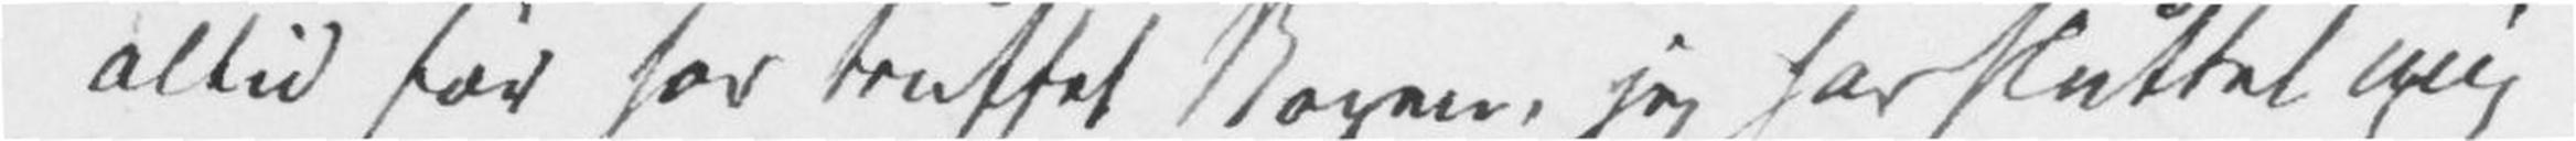altid før har truffet Nogen, jeg har sluttet mig
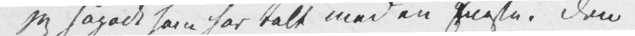jeg sågodt som har talt med en Eneste. Den
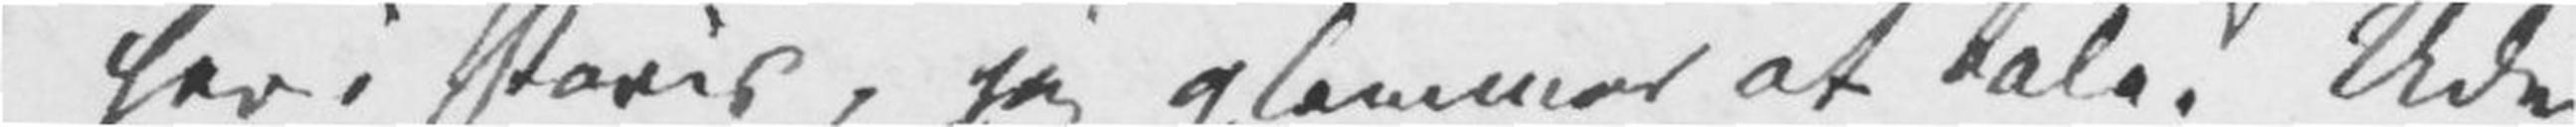her i Paris, jeg glemmer at tale. Ude
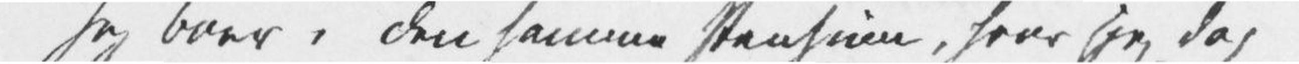Jeg boer i den samme Pension, hvor jeg dog
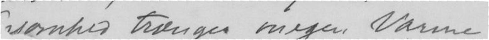Ensomhed trænger megen Varme
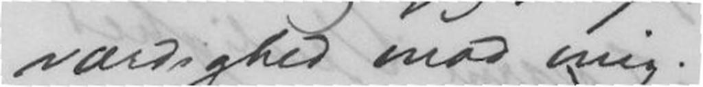værdighed mod mig.
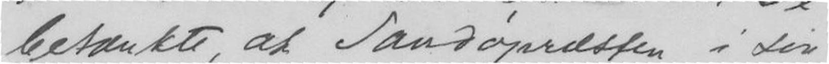betænkte, at Sandøpræsten i sin
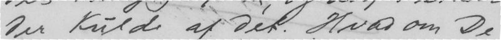der kulde af det. Hvar om De
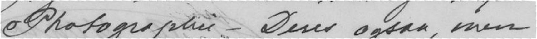Photographie - Deres ogsaa, men
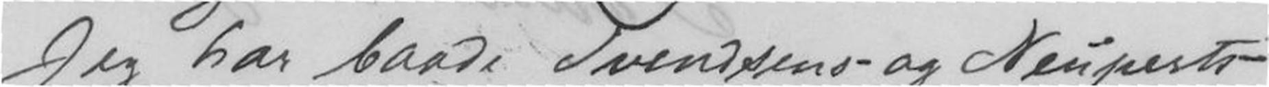Jeg har baade Svendsens- og Neuperts
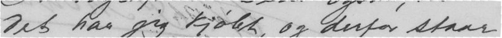det har jeg kjøbt, og derfor staar
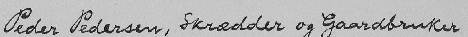Peder Pedersen, Skrædder og Gaardbruker
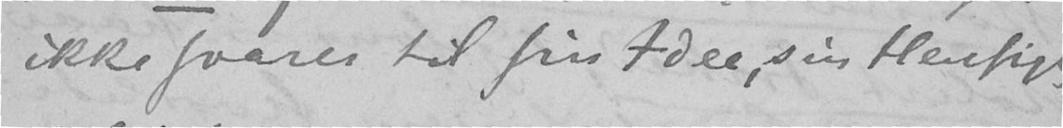ikke svarer til sin Idee, sin Hensigt
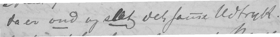da er ond og slet det samme Udtryk.
